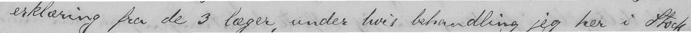erklæring fra de 3 læger, under hvis behandling jeg her i Stock-
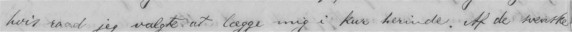hvis raad jeg valgte at lægge mig i kur herinde. Af de svenske
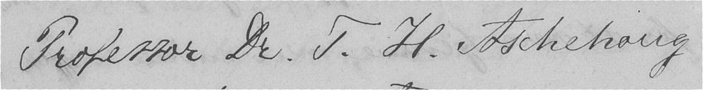Professor Dr. T. H. Aschehoug
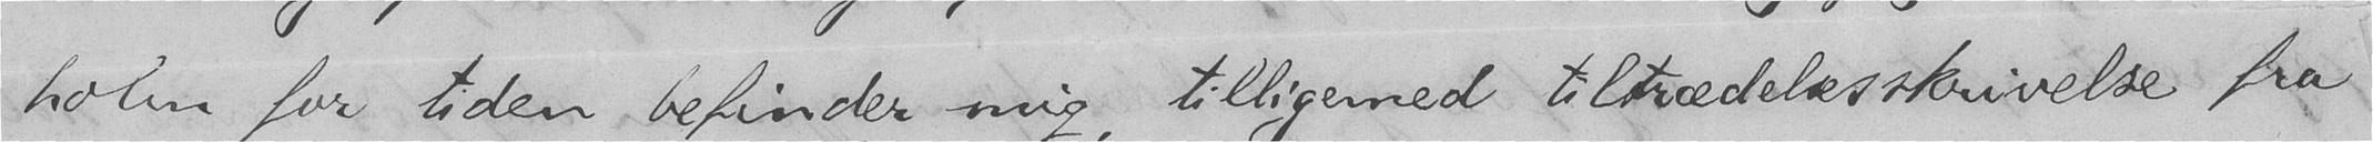holm for tiden befinder mig, tilligemed tiltrædelsesskrivelse fra
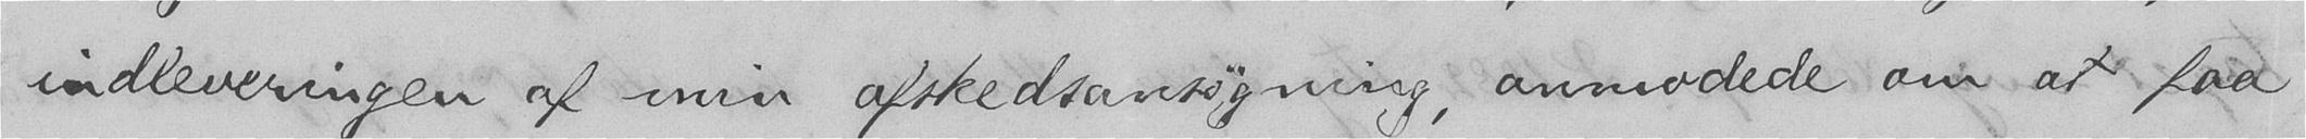indleveringen af min afskedsansøgning, anmodede om at faa
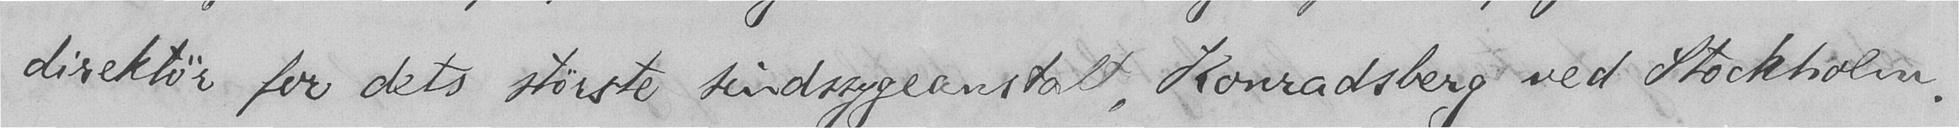direktør for dets største sindsygeanstalt, Konradsberg ved Stockholm.
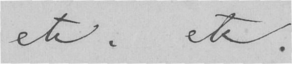etc. etc.
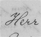Herr
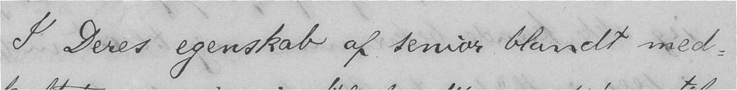I Deres egenskab af senior blandt med-
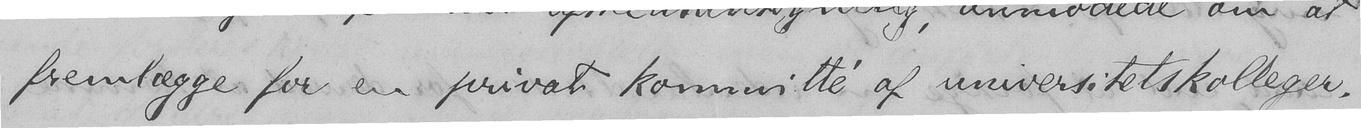fremlægge for en privat kommitté af universitetskolleger.
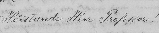Høistærede Herr Professor!
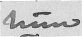hun
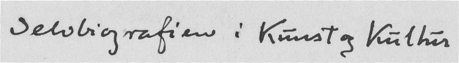Selvbiografien i Kunst og kultur
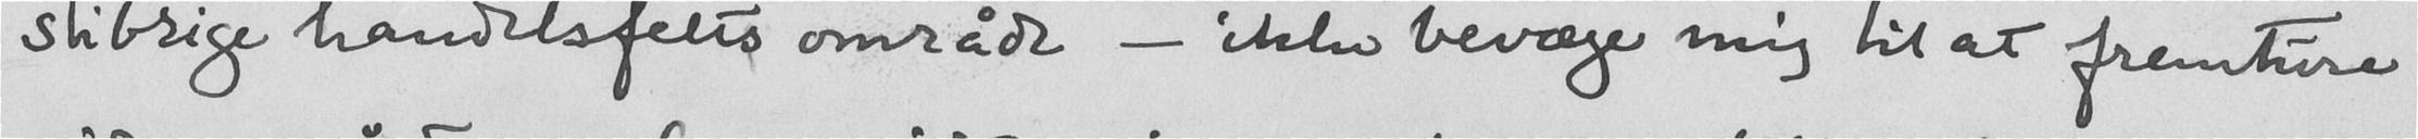slibrige handelsfelts område - ikke bevæge mig til at fremture
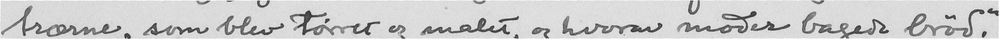trærne, som blev tørret og malet, og hvorav moder bagede brød."
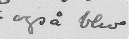også blev
